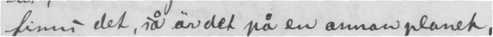finns det, så är det på en annan planet,
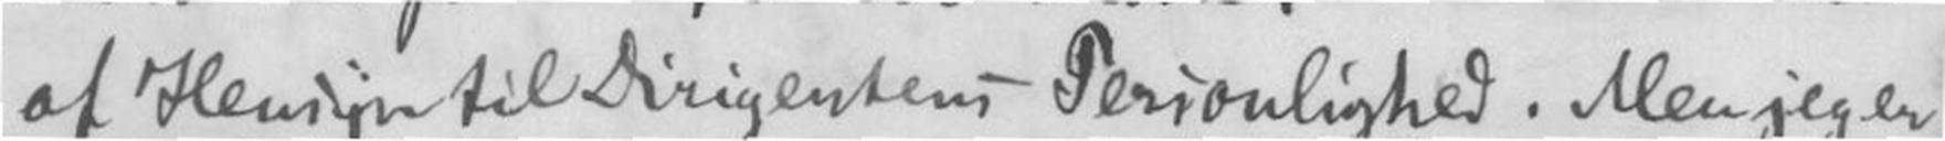af Hensyn til Dirigentens Personlighed. Men jeg er
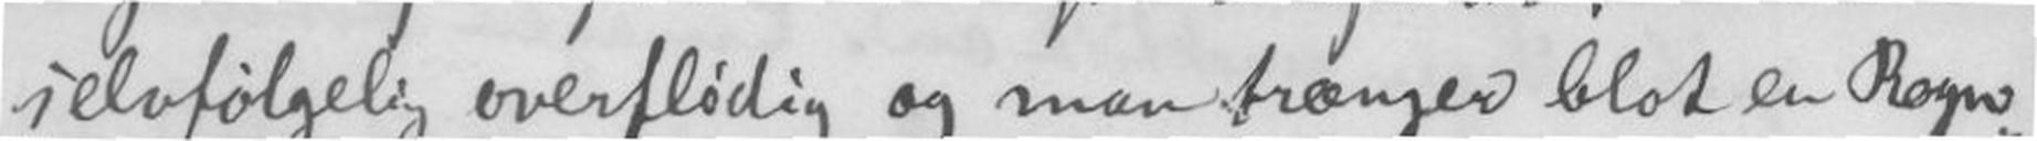selvfølgelig overflødig og man trænger blot en Regn-
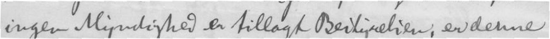ingen Myndighed er tillagt Bestyrelsen, er denne
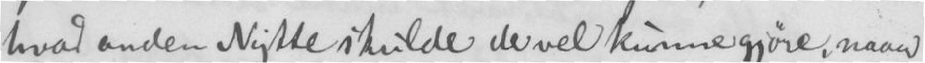hvad anden Nytte skulde de vel kunne gjøre, naar
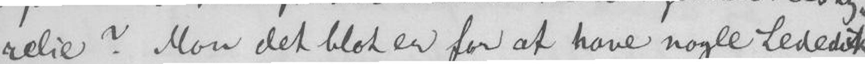relse? Mon det blot er for at have nogle Lededuk-
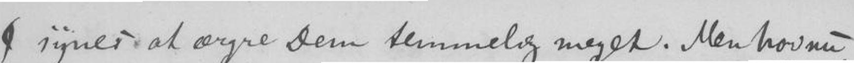I synes at ægre Dem temmelig meget. Men hav nu
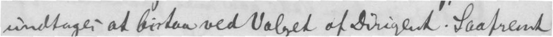undtages at bestaa ved Valget af Dirigent. Saafremt
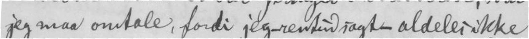jeg maa omtale, fordi jeg nesten sagt aldeles ikke
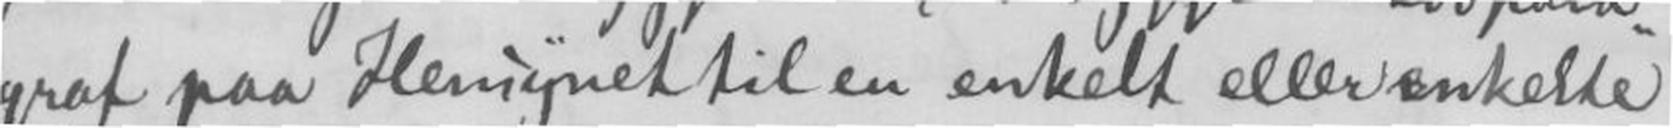ragraf paa Hensynet til en enkelt eller enkelte
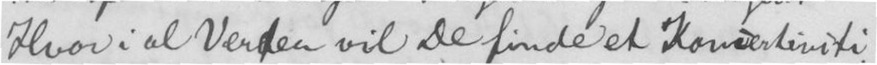Hvor i al Verden vil De finde et Konsertinsti-
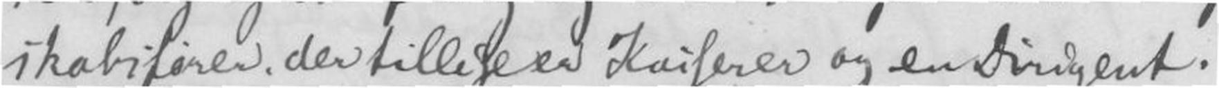skabsfører der tilligere er Kassarer og en Dirigent.
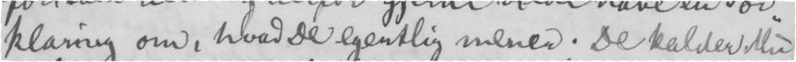klaring om, hvad De egentlig mener. De kalder Mu-
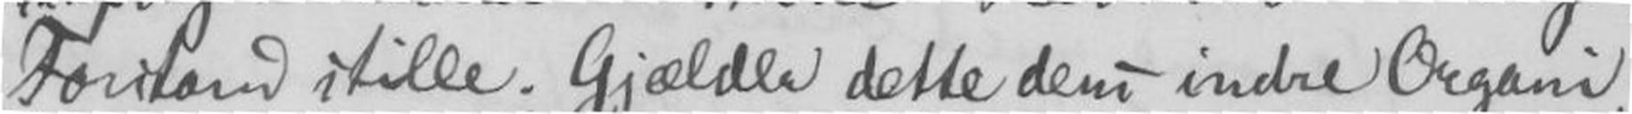Forstand stille. Gjælder dette dens indre Orgeni-
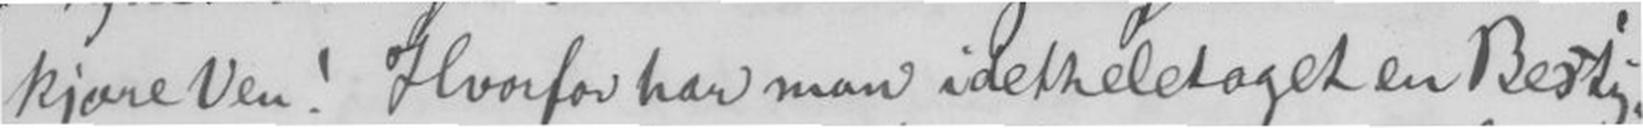kjære Ven! Hvorfor har man idetheletaget en Besty
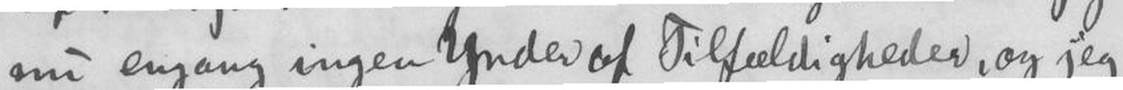nu engang ingen Ynder af Tilfeldigheder, og jeg
